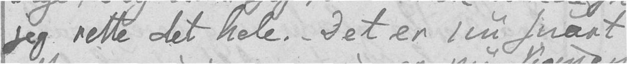jeg rette det hele. Det er nu snart
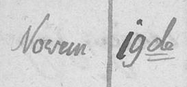Novem 19de
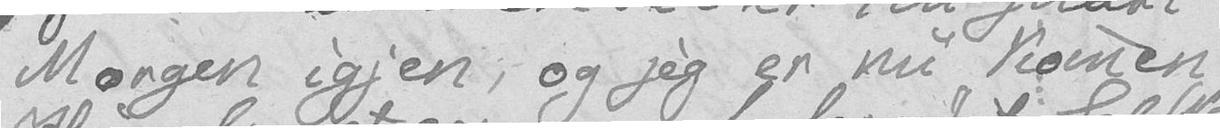Morgen igjen, og jeg er nu kommen
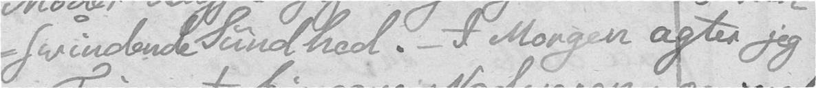-svindende Sundhed. – I Morgen agter jeg
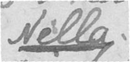Nella.
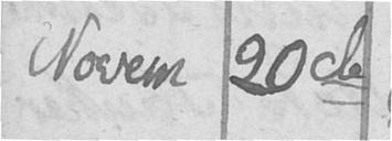Novem 20de
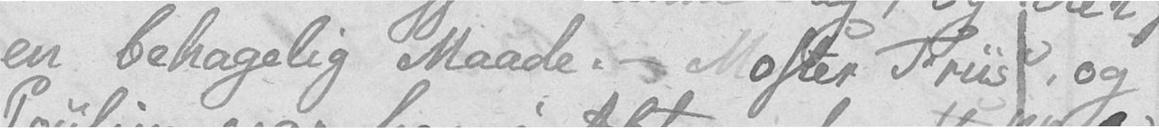en behagelig Maade. – Moster Friis, og
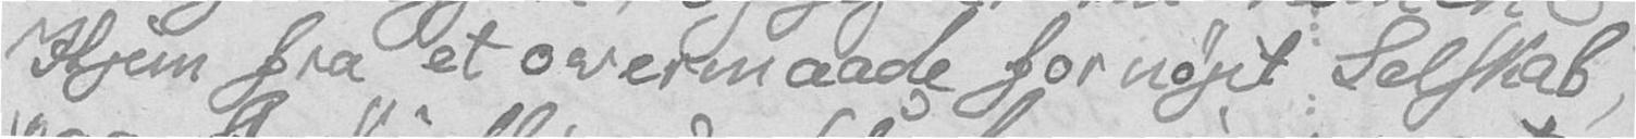Hjem fra et overmaade fornøjet Selskab,
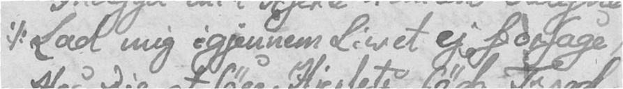:/: Lad mig igjennem Livet ej forsage
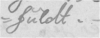-fuldt. –
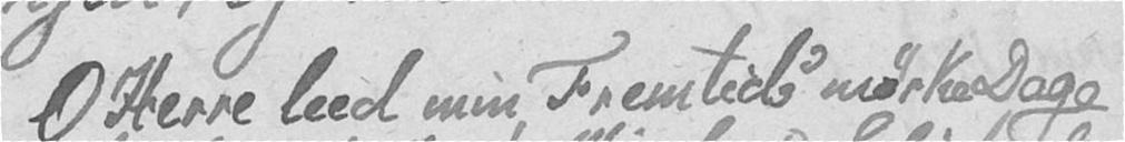O Herre leed min Fremtids mørke Dage
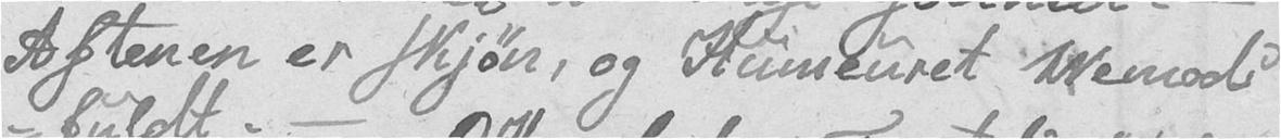Aftenen er skjøn, og Humeuret Vemods
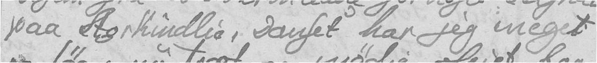paa Storkindlie, Danset har jeg meget
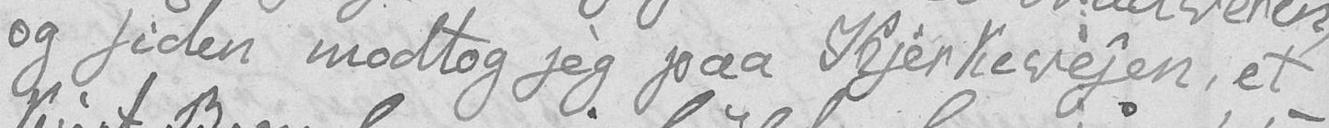og siden modtog jeg paa Kjerkevejen, et
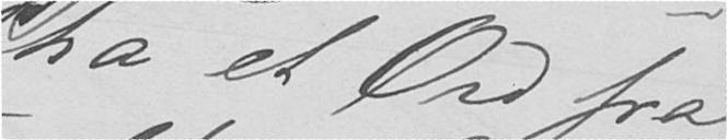ha et Ord fra
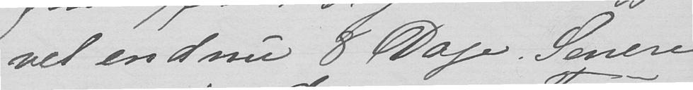vel endnu 8 Dage. Senere
